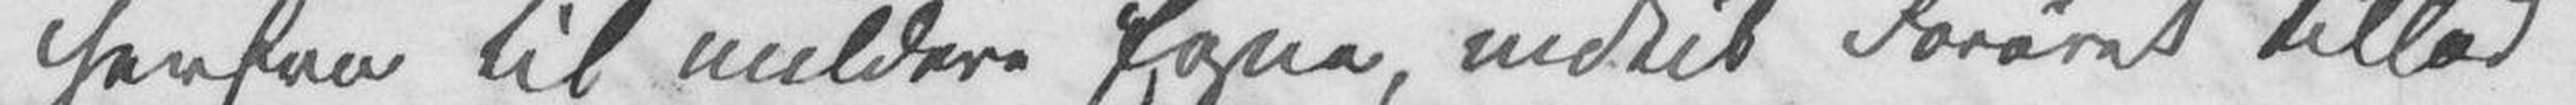herfra til mildere Egne, indtil Foråret tillod
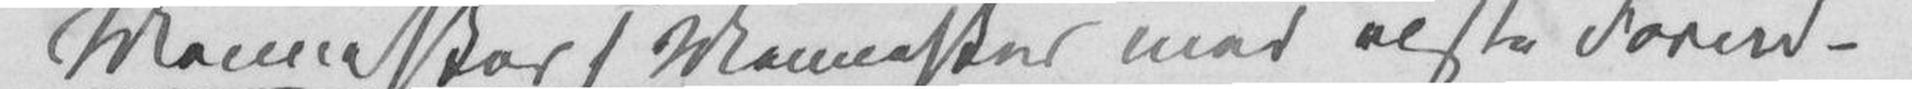Mennesker (Mennesker med visse Forud¬
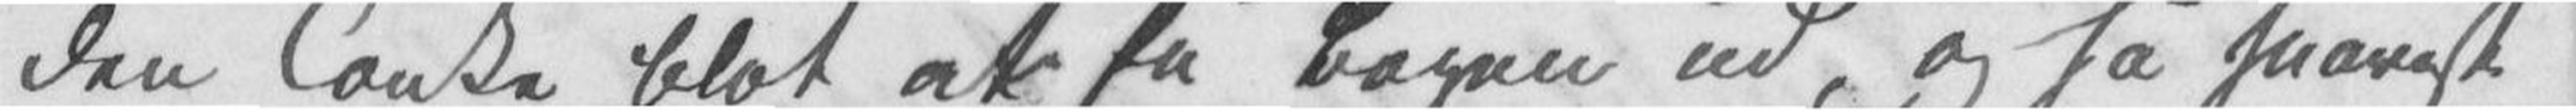den Tanke blot at få Bogen ud, og så snarest
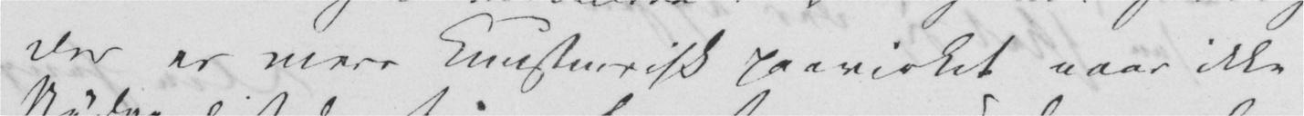der er mere kunstnerisk paavirket naar ikke
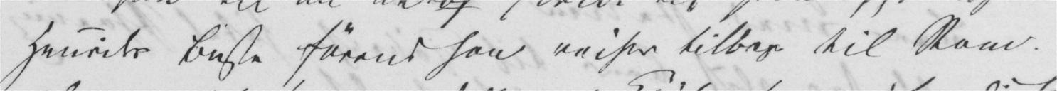Henriks Buste førend han reiser tilbage til Rom.
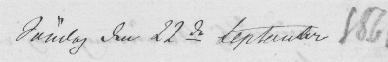Søndag den 22de September
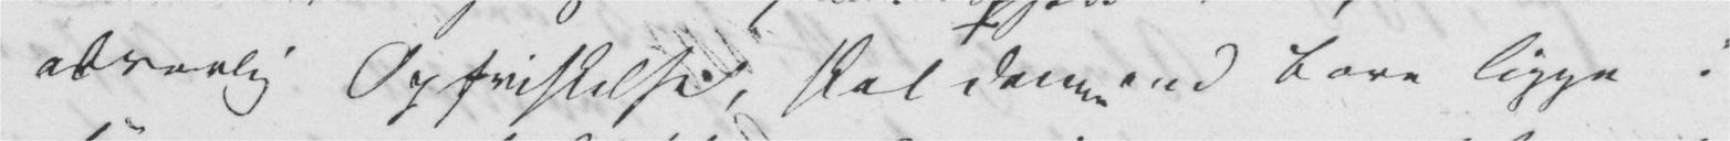alvorlig Opfriskelse, skal denne end bare ligge i
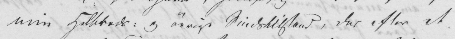min Helbreds: og øvrige Sindstilstand, der efter et
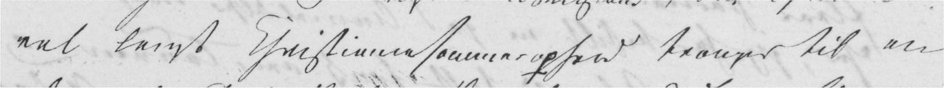vel langt Christianiasommerophold trænger til en
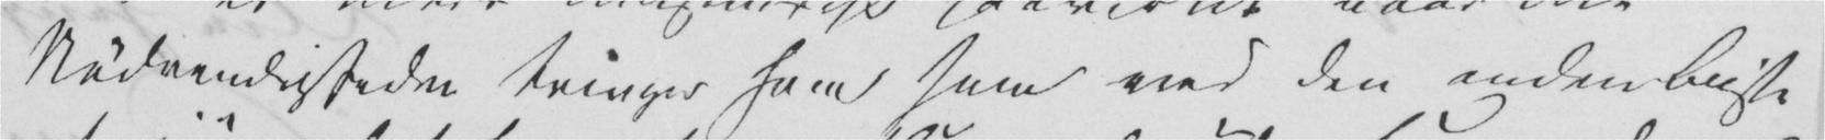Nødvendigheden tvinger ham som ved den anden Buste
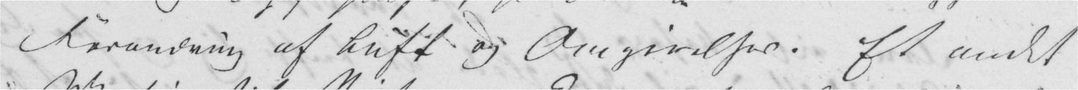Forandring af Luft og Omgivelser. Et andet
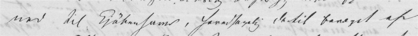ned til Kjøbenhavn, hovedsagelig dertil bevæget af
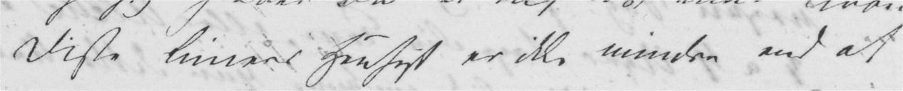Disse Liniers Hensigt er ikke mindre end at
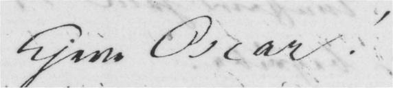Kjere Oscar!
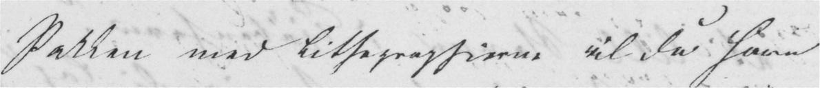Pakken med Lithographierne vil Du have
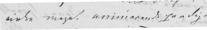virke meget animerende paa Dig.
